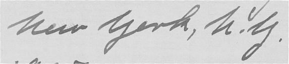Nen fjork, N.Y.
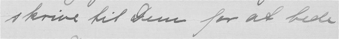skrive til Dem for at bede
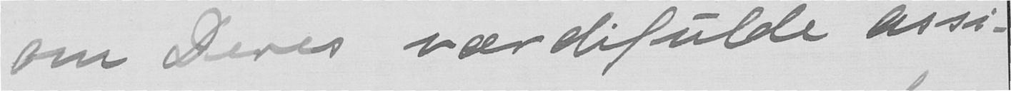om Deres værdifulde assi-
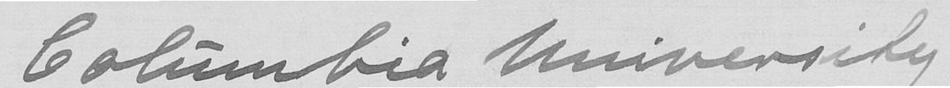Columbia Universitet
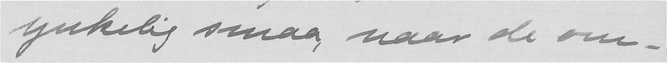ynkelig smaa naar de om-
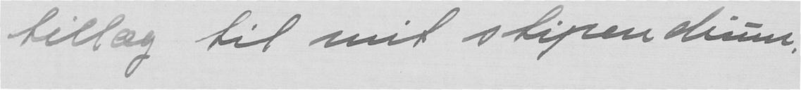tillæg til mit stipendium.
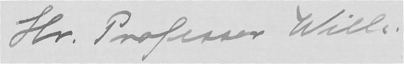Hr. Professor Wille!
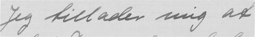Jeg tillader mig at
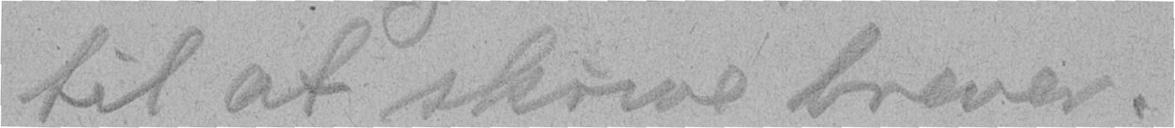til at skrive brever.
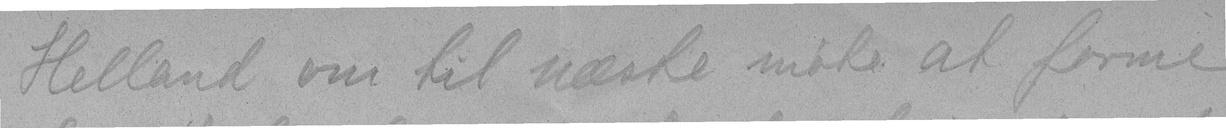Helland om til næste møte at forme
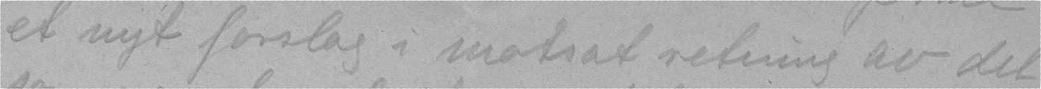et nyt forslag i motsat retning av det
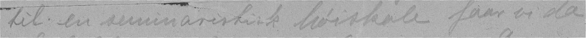til en seminaristisk høiskole faar vi da
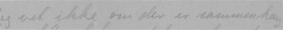Jeg vet ikke om der er sammenheng
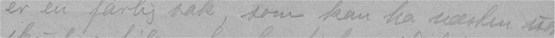er en farlig sak, som kan ha næsten uover-
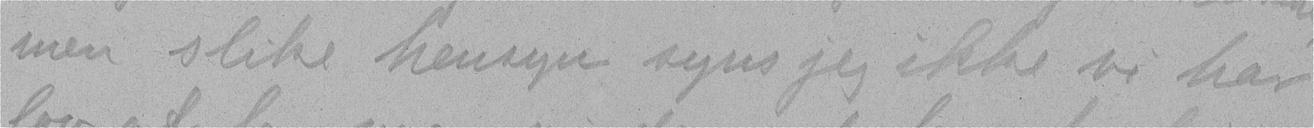men slike hensyn syns jeg ikke vi har
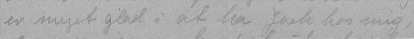er meget glad i at ha Jack hos mig, og,
